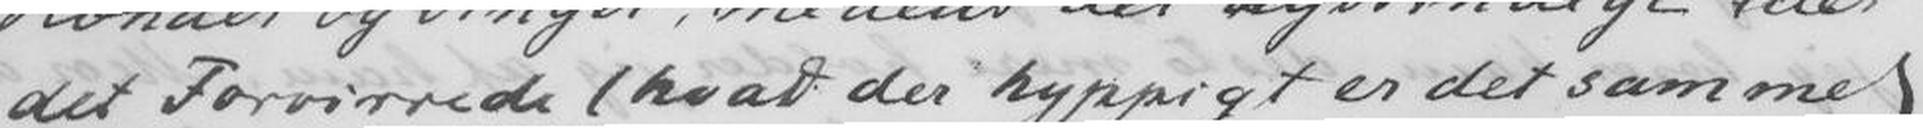det Forvirrede (hvad der hyppigt er det samme)
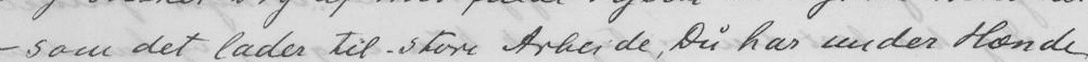- som det lader til store Arbeide, Du har under Hænde
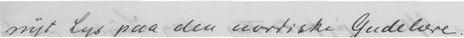nyt Lys paa den nordiske Gudelære.
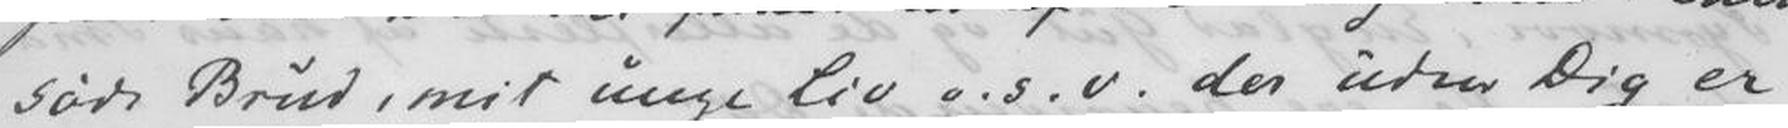søde Brud, mit unge Liv o.s.v. der uden Dig er
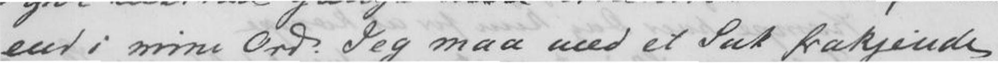end i mine Ord. Jeg maa med et Suk frakjende
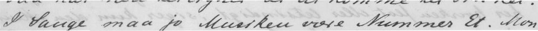I Sange maa jo Musiken være Nummer Et. Mon
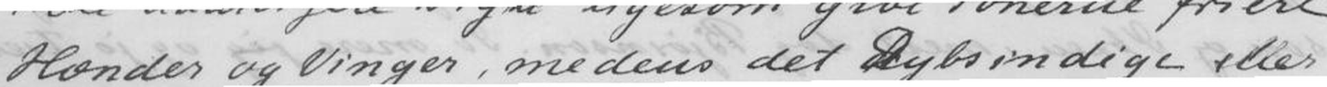Hænder og Vinger, medens det Dybsindige sker
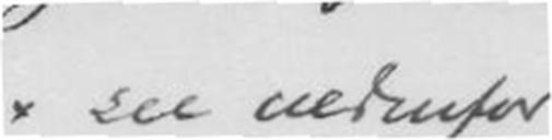x see nedenfor
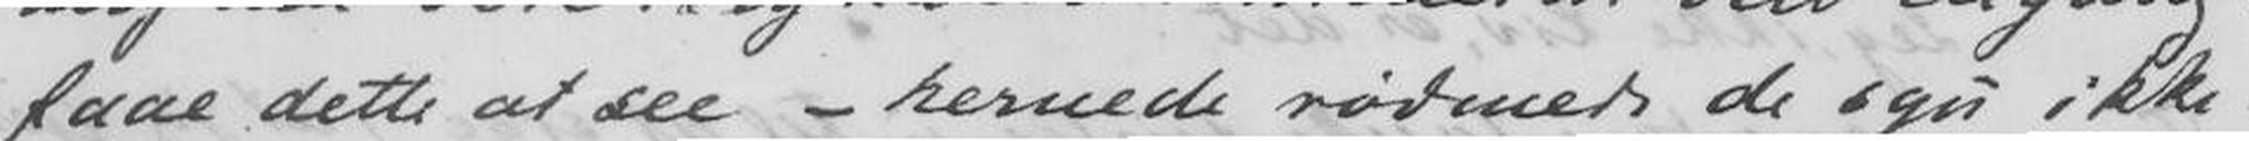faae dette at see - hernede rødmede de sgu ikke -
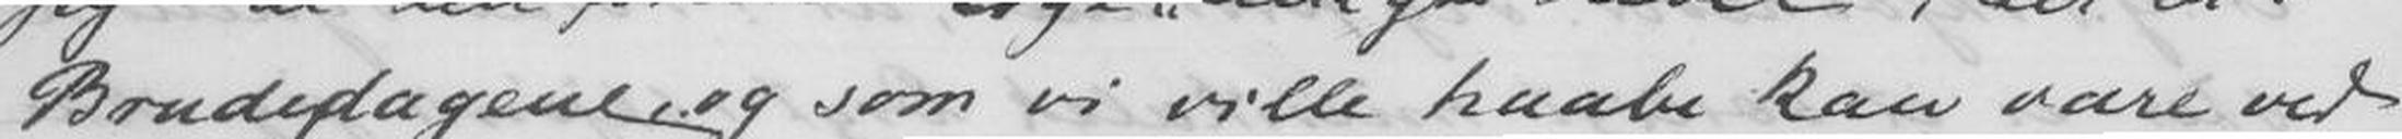Brudeplagene, og som vi ville haabe kan være ved
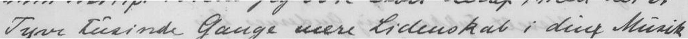Tyve tusinde Gange mere Lidenskab i Din Musik
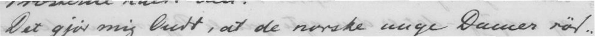Det gjør mig Andet, at de norske unge Damer rød-
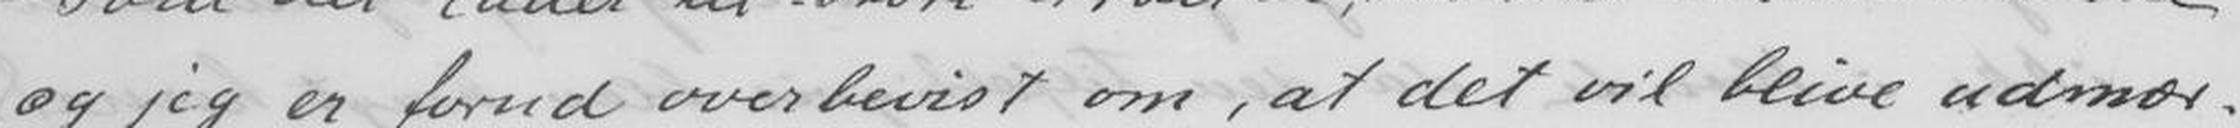og jeg er forud overbevist om, at det vil blive udmær-
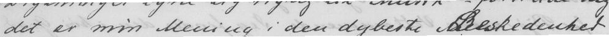det er min Mening i den dubeste Beskedenhed
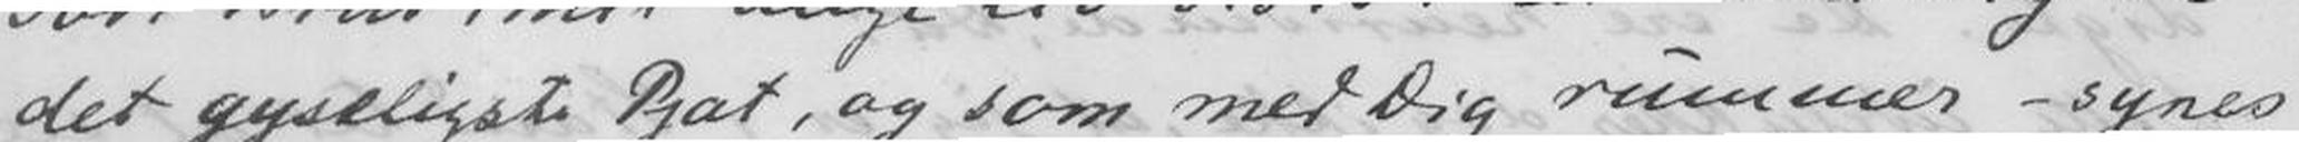det gyseligste Pjat, og som med Dig rummer - synes
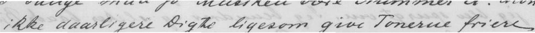ikke daarligere Digte ligesom give Tonerne friere
